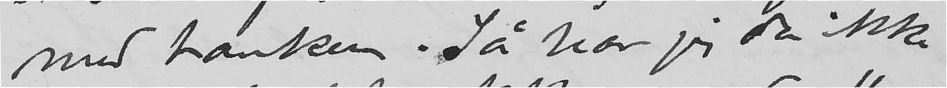med tanken. Så har jeg da ikke
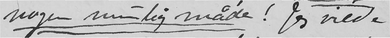nogen mulig måde! Jeg vilde
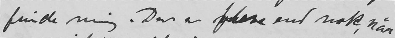finde mig. Den er flere end nok, når
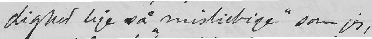dighed lige så "misliebige" som jeg,
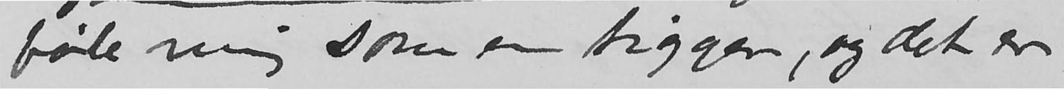føle mig som en tigger, og det er
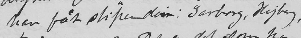har fåt stipendium: Garborg,Hejberg,
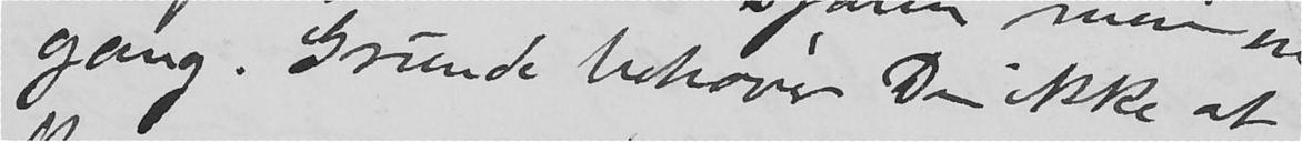gang. Grunde behøver Du ikke at
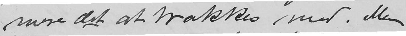mere det at trækkes med. Men
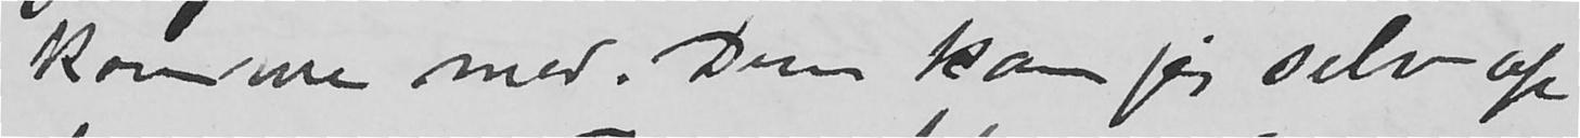komme med. Dem kan jeg selv op-
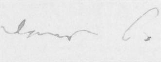Deres C.
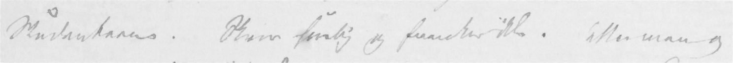Studenterne. Skriv hurtig og franker ikke. Dette maa og
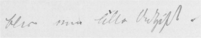blive min lille Udgift.
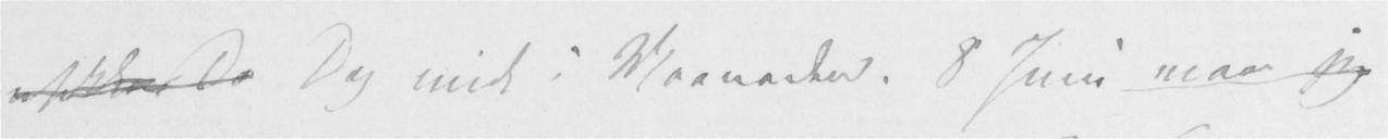usikker Da Dag midt i Maaneden. 8 Juni maa jeg
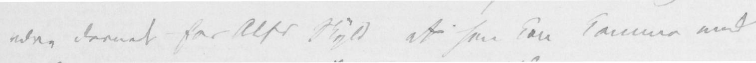være dernede for Alfs Skyld at han kan komme med
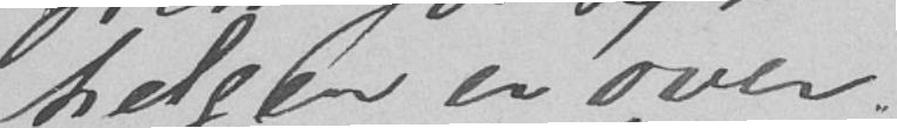helgen er over.
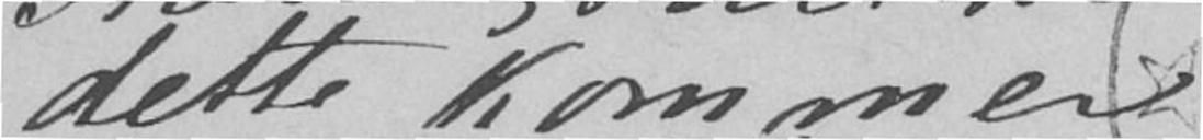dette kommer
